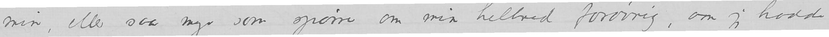min, eller saa mye som spørre om min helbred for øvrig, om jeg hadde
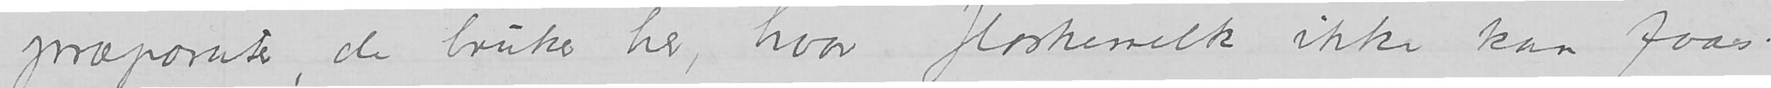præparater, de bruker her, hvor flaskemelk ikke kan faas.
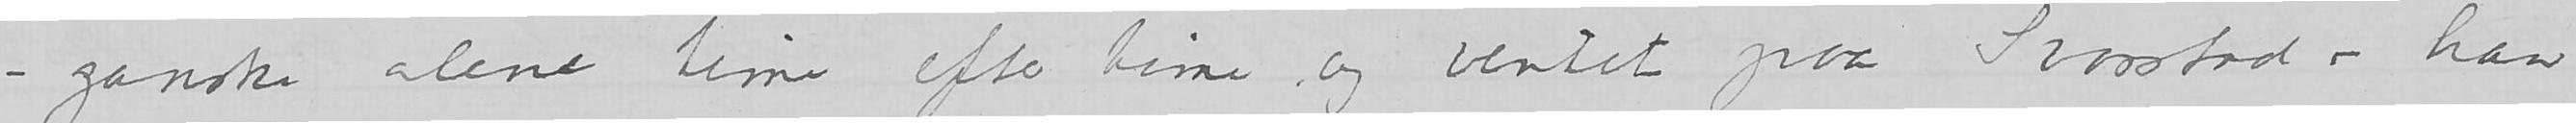– ganske alene time efter time og ventet paa Svarstad – han
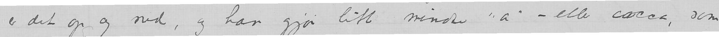er det op og ned, og han gjør litt mindre «a» - eller cacca som
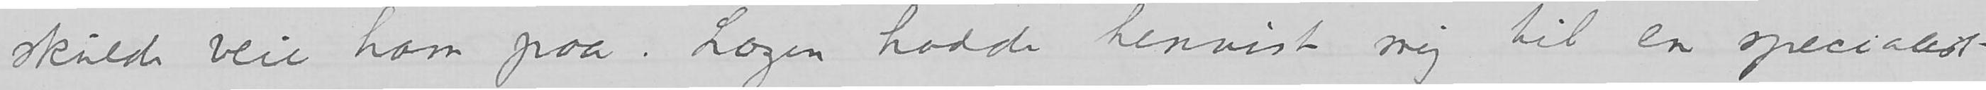skulde veie ham paa. Lægen hadde henvist mig til en specialist
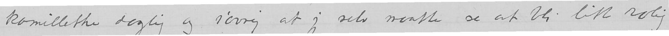kamillethe daglig og iøvrig at jeg selv maatte se at bli litt rolig
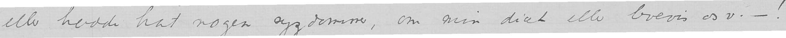eller hadde hat nogen sygdommer, om min diæt eller levevis osv. –!
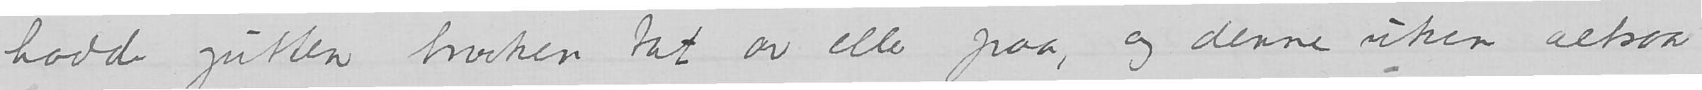hadde gutten hverken tat av eller paa, og denne uken altsaa
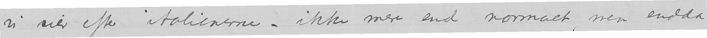vi sier efter italierne – ikke mere end normalt, men endda
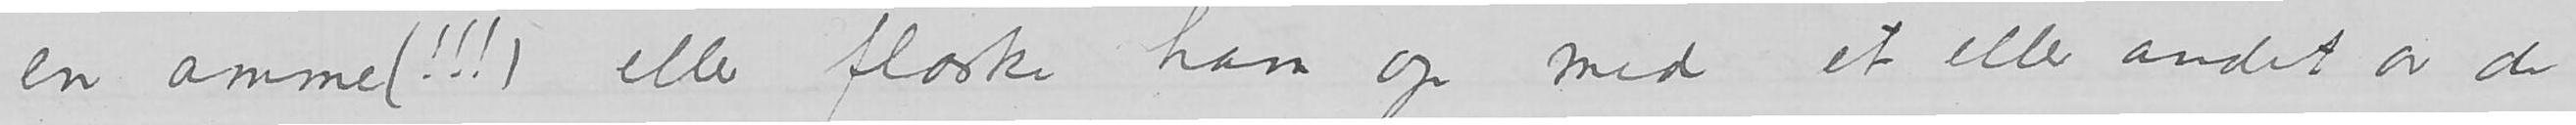en amme (!!!) eller flaske ham op med et eller andet av de
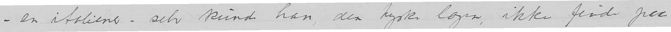– en italiener – selv kunde han, den tyske lægen, ikke finde paa
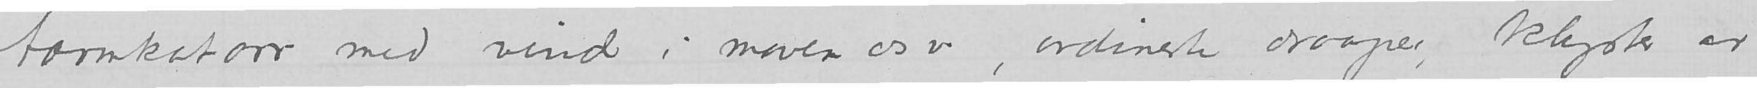tarmkatarr med vind i magen osv, ordinerte draaper, klyster av
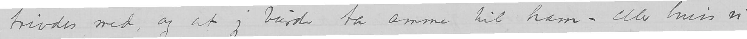trivdes med, og at jeg burde ta amme til ham – eller hvis vi
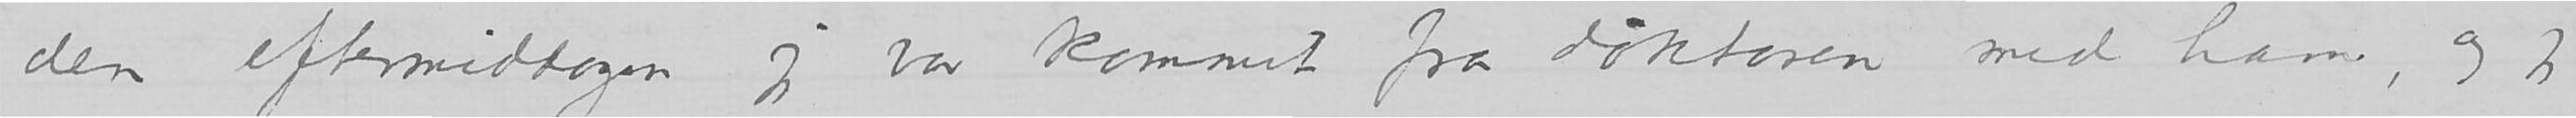den eftermiddagen jeg var kommet fra doktoren med ham, og jeg
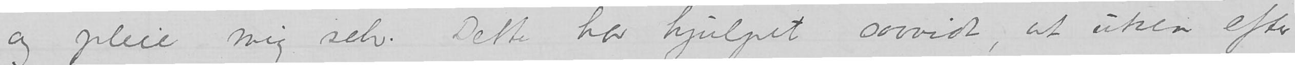og pleie mig selv. Dette har hjulpet saavidt, at uken efter
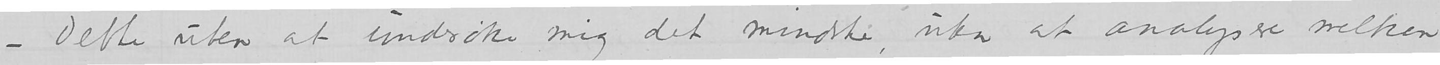– Dette uten at undersøke mig det mindste, uten at analysere melken
